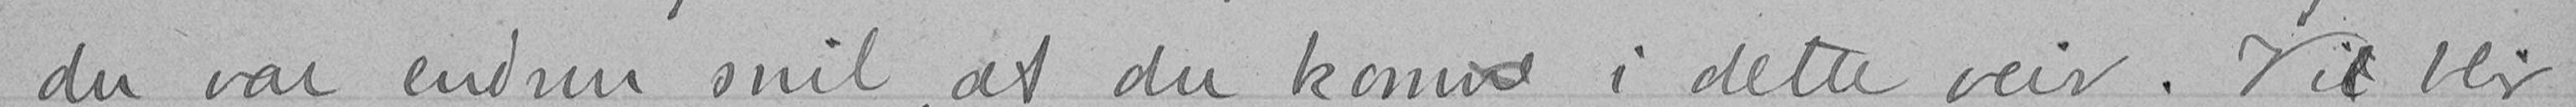du var endnu snil, at du komne i dette veir. Vil blir
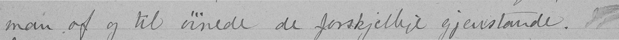man af og til øinede de forskjellige gjenstande. Jo
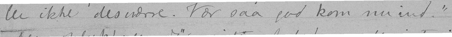ler ikke desværre. Vær saa god kom nu ind."
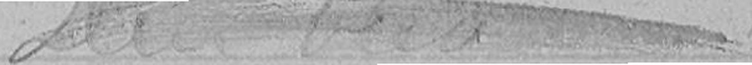Det blev
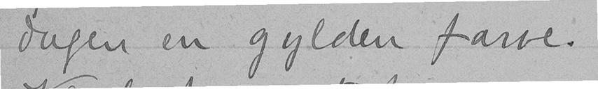dugen en gylden farve.
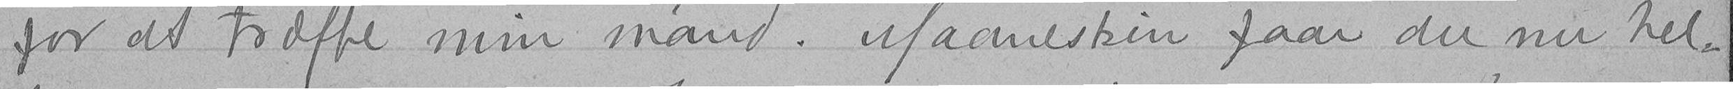for at træffe min mand. Maaneskin faar du nu hel-
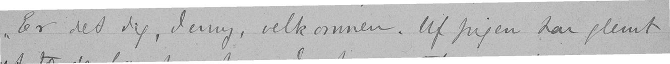"Er det dig, Jenny, velkommen. Uf pigen har glemt
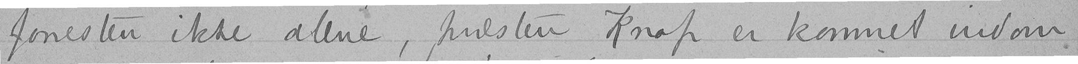forresten ikke alene, præsten Knop er kommet indom
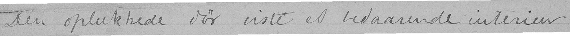Den oplukkede dør viste et bedaarende interieur
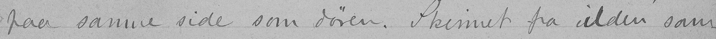paa samme side som døren. Skinnet fra ilden sam-
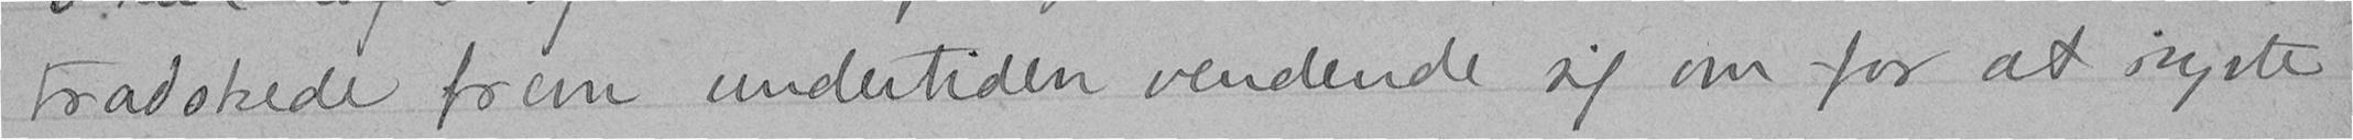tradskede frem undertiden vendende sig om for at ryste
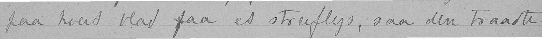paa hvert blad laa et streiflys, saa den traadte
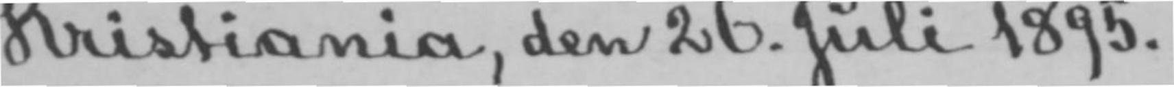Kristiania, den 26. Juli 1895.
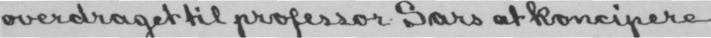overdraget til professor Sars at koncipere
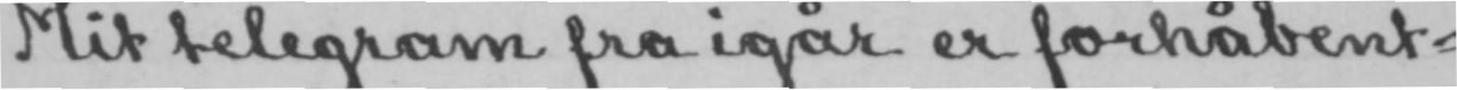Mit telegram fra igår er forhåbent-
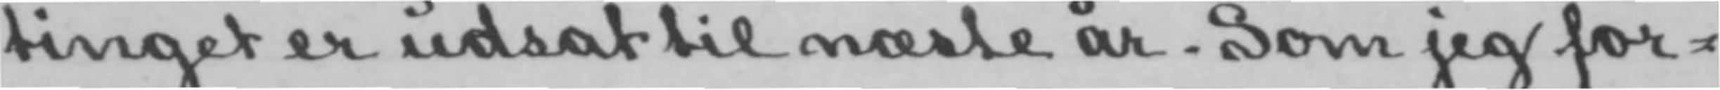tinget er udsat til næste år. Som jeg for-
